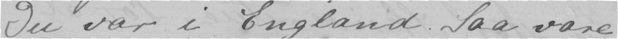Du var i England. Saa vare
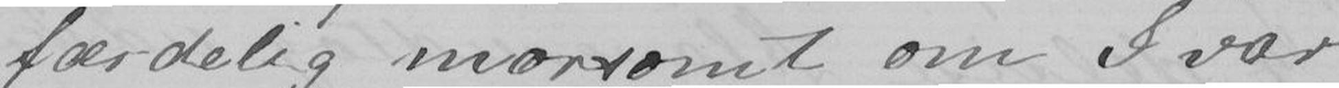færdelig morsomt om I var
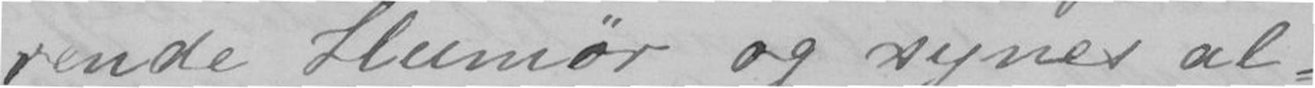rende Humør og synes al-
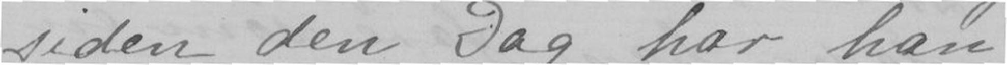siden den Dag har han
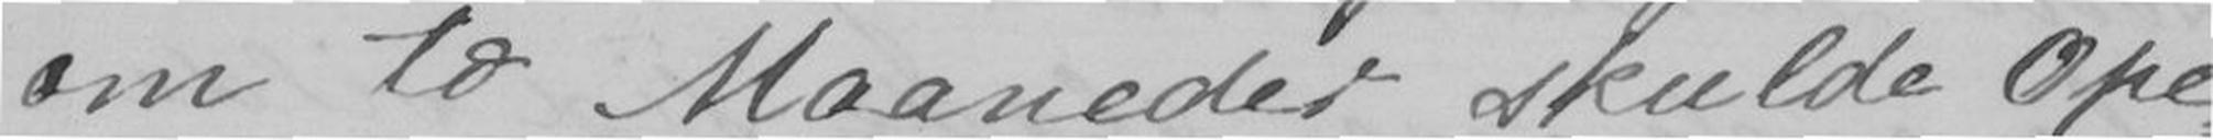om to Maaneder skulde Ope-
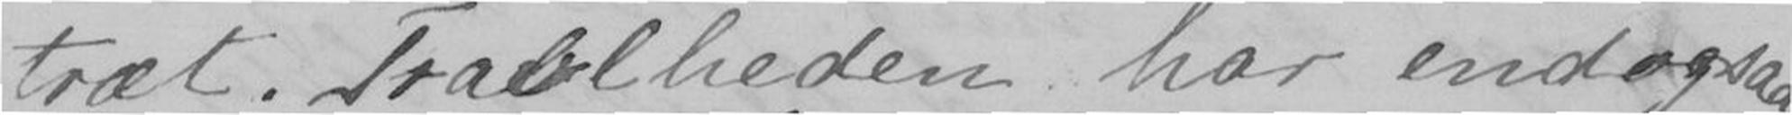træt. Travlheden har endogsaa
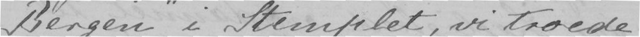Bergen i Stemplet, vi troede
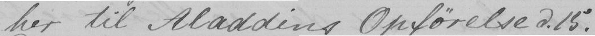her til Aladdins Opførelse d.15.
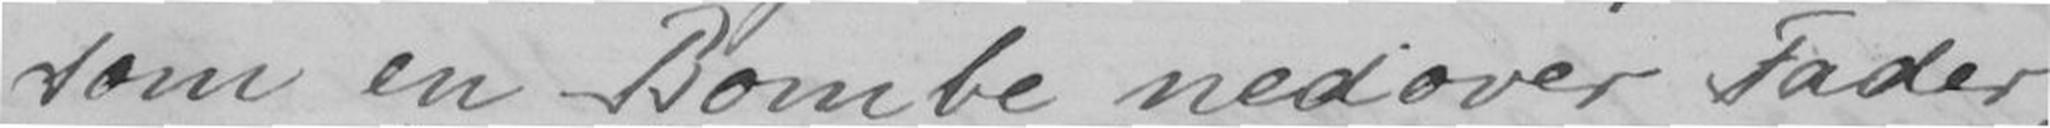som en Bombe nedover Fader,
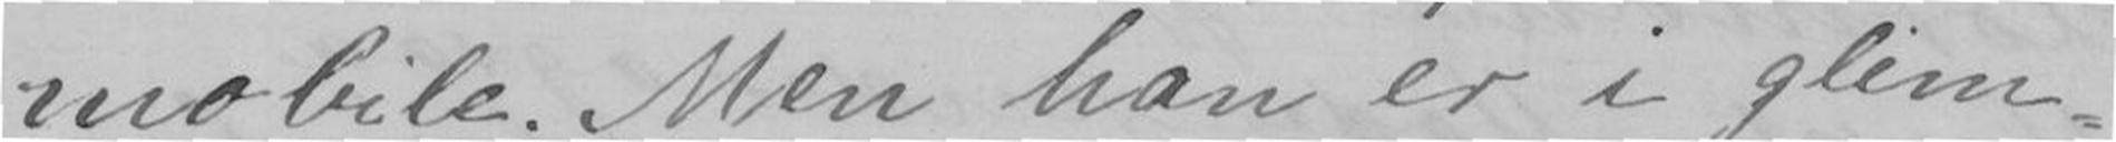mobile. Men han er i glim-
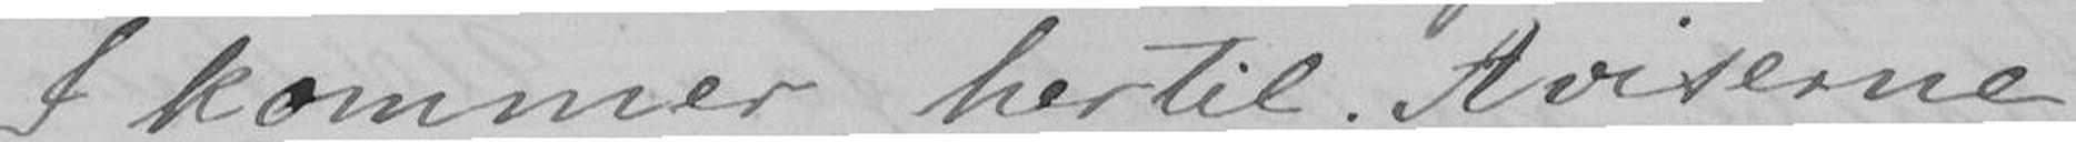I kommer hertil. Aviserne
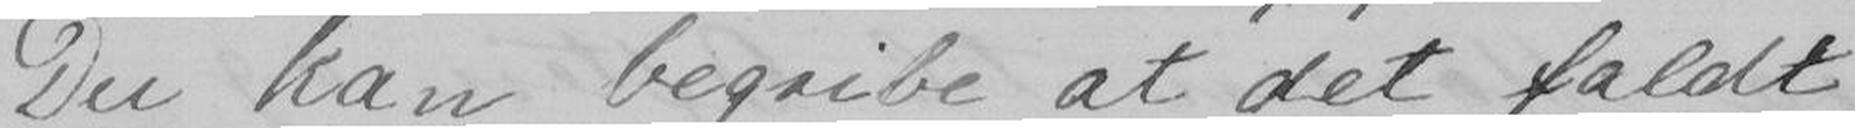Du kan begribe at det faldt
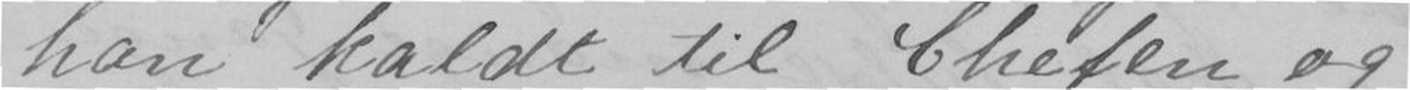han kaldt til Chefen og
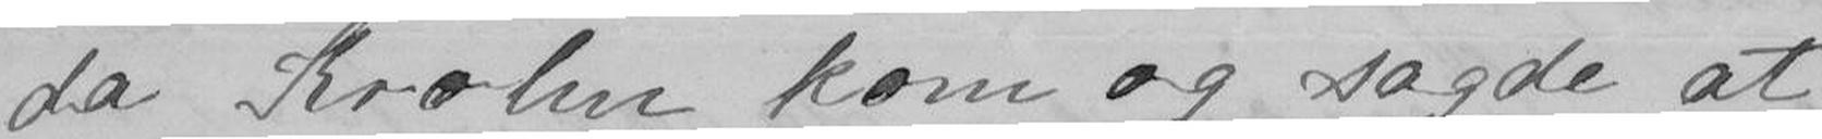da Krohn kom og sagde at
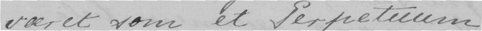været som et Perpetuum
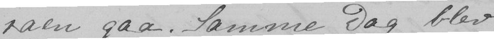raen gaa. Samme Dag blev
